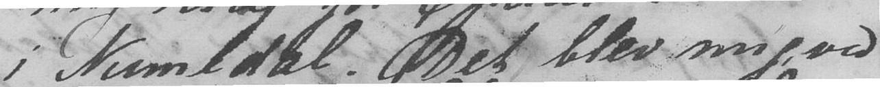i Numedal. Det blev mig ved
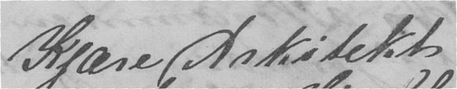Kjære Arkitekt
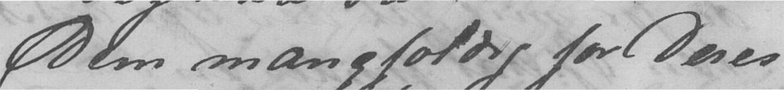Dem mangfoldig for Deres
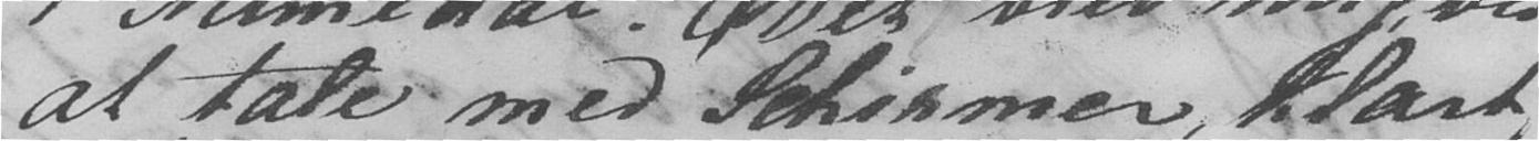at tale med Schirmer klart,
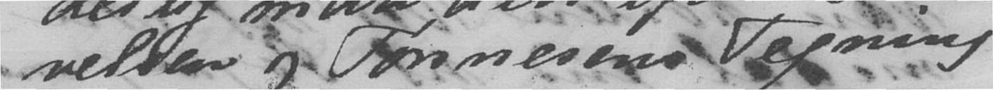velsen og Tønnesens Tegning
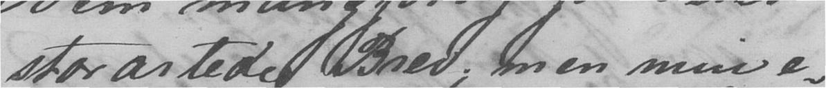storartede Brev, men min e-
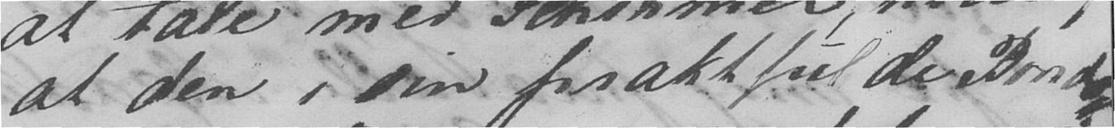at den i sin praktfulde Bondak-
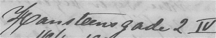Hansteensgade 2 IV
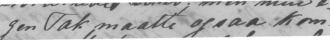gen Tak maatte ogsaa kom-
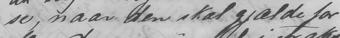se, naar den skal gjælde for
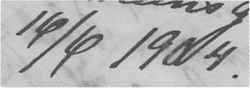16/6 1904.
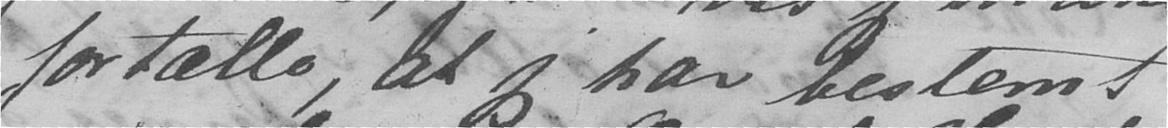fortælle, at jeg har bestemt
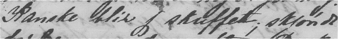Kanske blir jeg skuffet; skjøndt
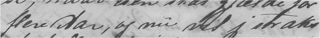flere Aar, og nu vil jeg straks
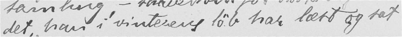det, han i vinterens løb har læst og sat
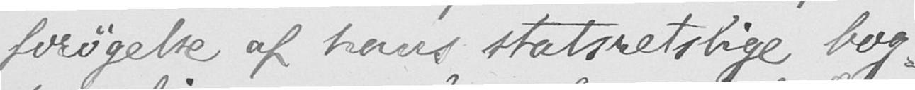forøgelse af hans statsretslige bog-
